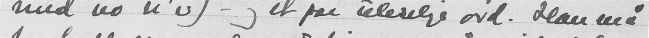med to n'er) - og et par uleselige ord. Han må
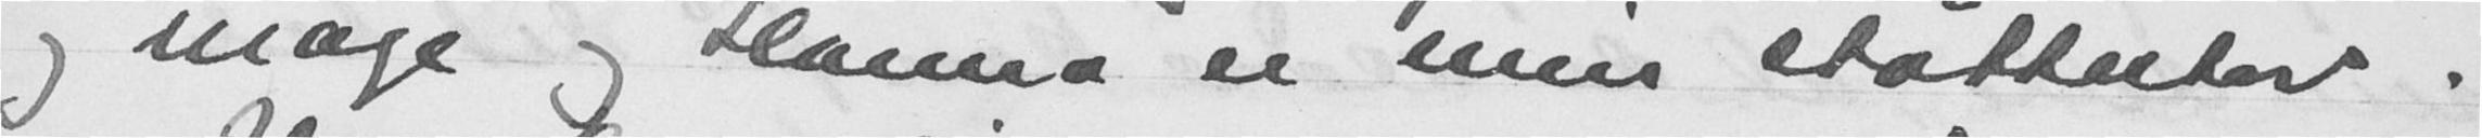og mage og Hanna er min støttestor.
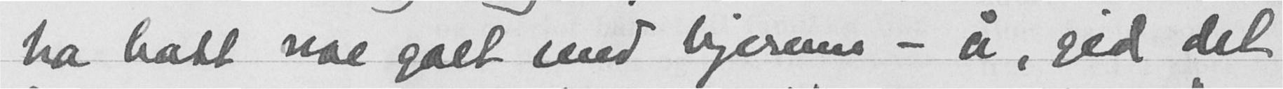ha hatt noe galt med hjernen - å, gid det
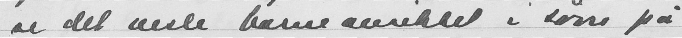se det vesle barneansiktet i søvn på
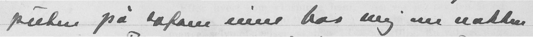puten på sofaen min hos mig om natten.
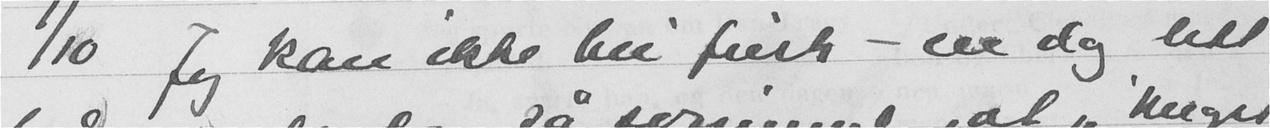1/10 Jeg kan ikke bli frisk - en dag litt
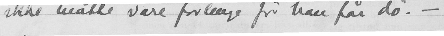ikke måtte være forlenge før han får dø. -
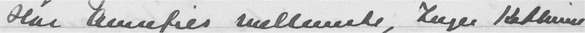Har Amrefries mellomste, Inger Kotheim
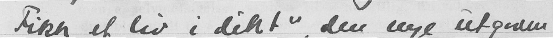Fikk "et liv i dikt", den nye utgaven,
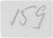158
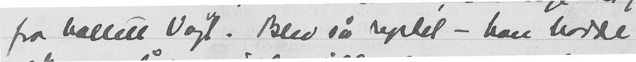fra Collett Vogt. Blev så reptel - han hadde
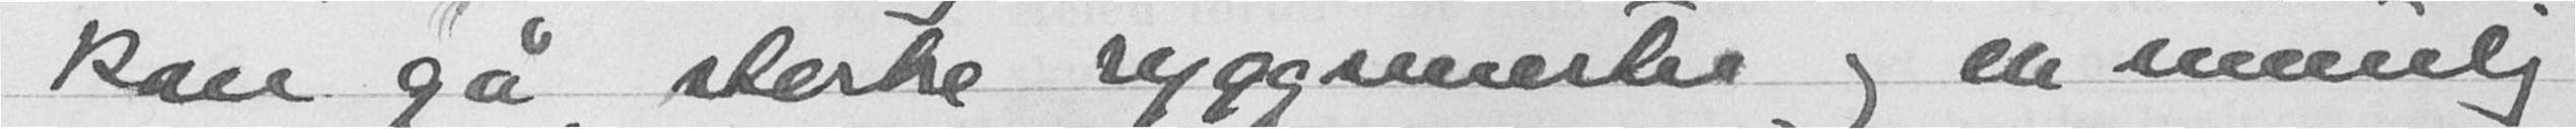kan gå, sterke ryggsmerter og en umulig
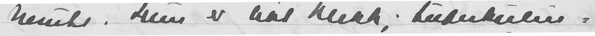herute. Hun er lat klekk; tuberkulose-
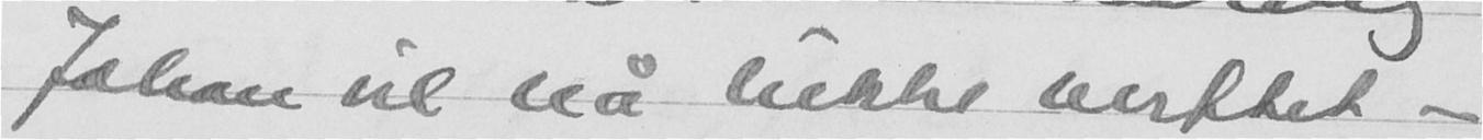Johan vil nå lukke verftet -
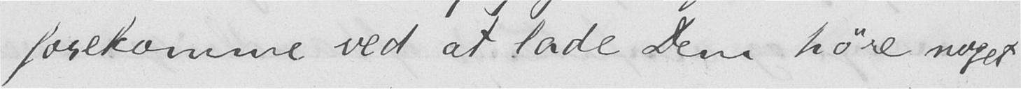forekomme ved at lade Dem høre noget
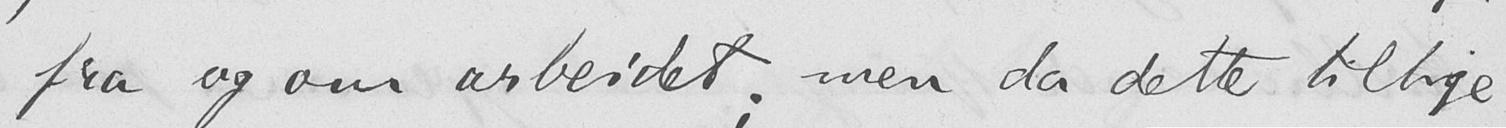fra og om arbeidet; men da dette tillige
